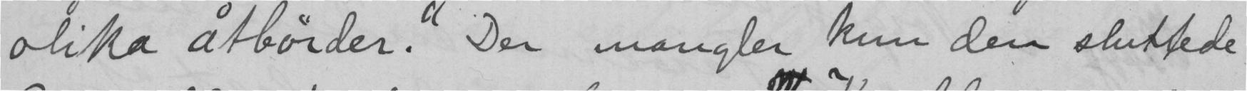olika åtbørder." Der mangler kun den sluttede
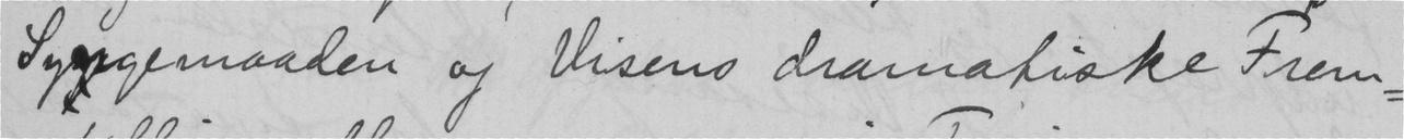Syngemaaden og Visens dramatiske Frem-
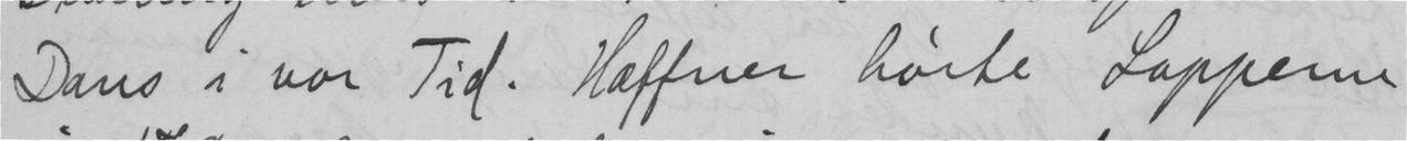Dans i vor Tid. Haffner hørte Lapperne
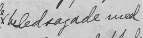beledsagade med
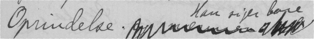Oprindelse.
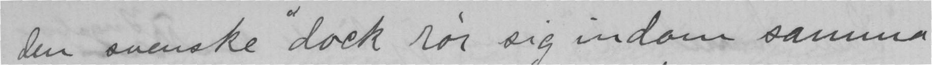den svenske "dock rør sig indom samma
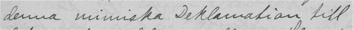denna mimiska Deklamation till
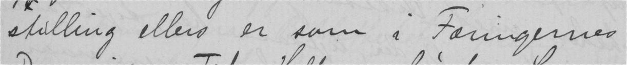stilling ellers er som i Færingernes
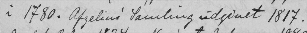i 1780. Afzelius' Samling udgivet 1817.
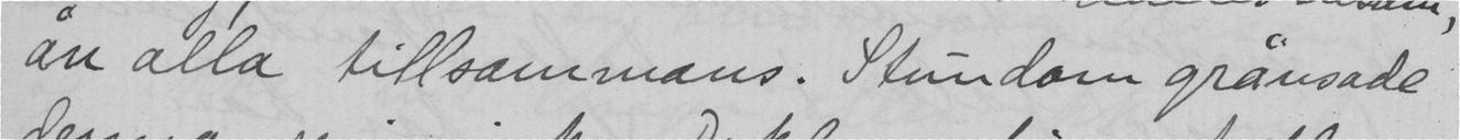än alla tillsammans. Stundom gränsade
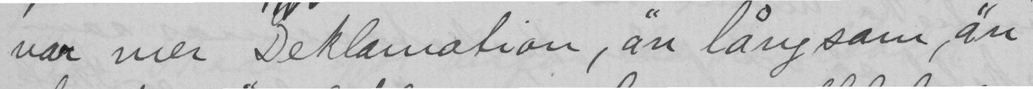var mer Deklamation, än lång som, än
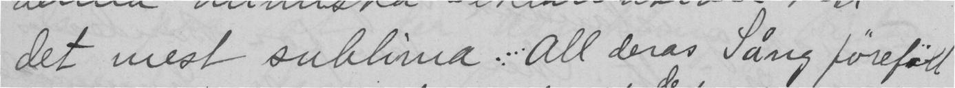det mest sublima: All deras Sång førefäll
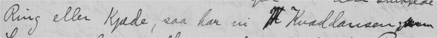Ring eller Kjæde, saa har vi  Kvaddansen;
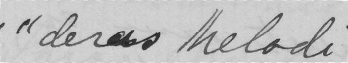"deres Melodi
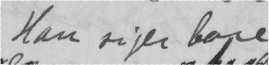Han siger bare
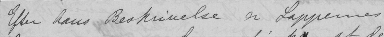Efter hans Beskrivelse er Lappernes
